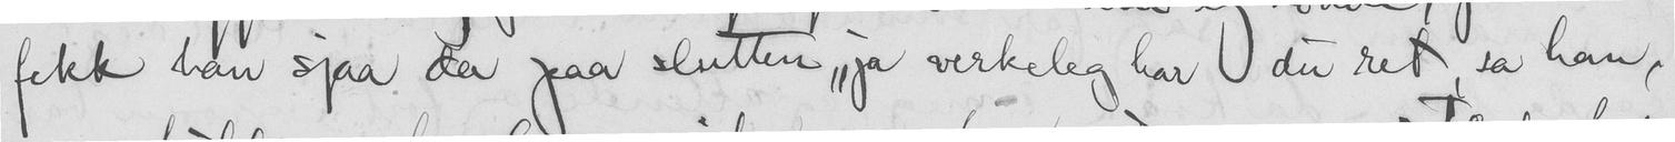fekk han sjaa de paa slutten "ja verkelig har du ret, sa han,
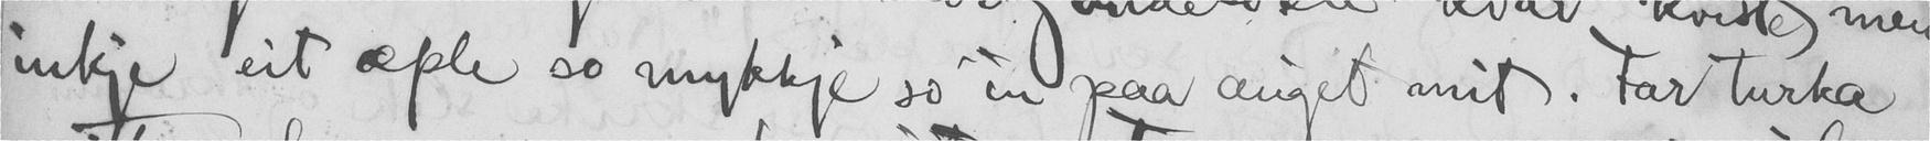inkje eit æple so mykkje so in paa auget mit . Far turka
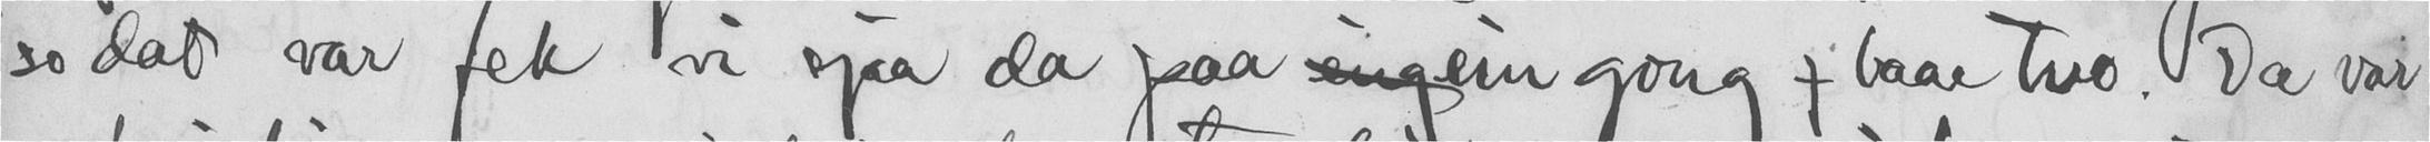so dat var fek vi sjaa da paa  ein gong  baae tvo. Da var
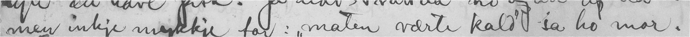men inkje mykkje for: "maten værte kald" sa ho mor.
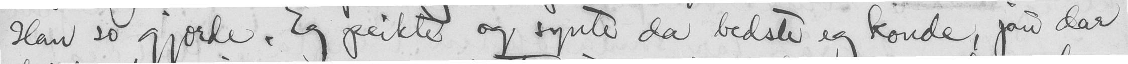Han so gjorde. Eg peikte og synte da bedste og konde, jau dar
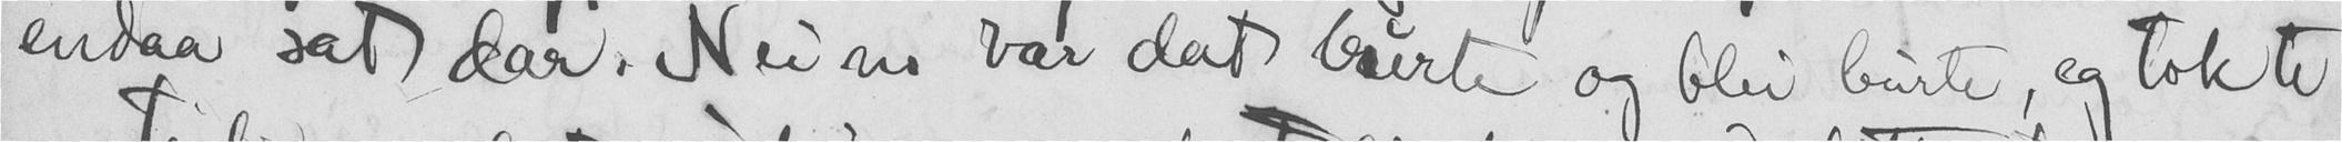endaa sat dar. Nei nu var det burte og blei burte, eg tok te
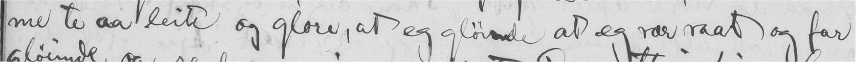me te aa leite og glore, at eg glømde at eg var vaat og far
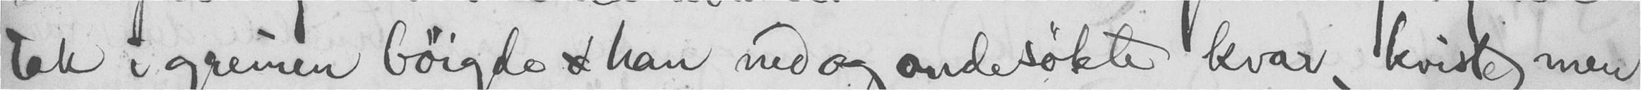tak i greinen bøigde  han ned og andesøkte kvar kvist men
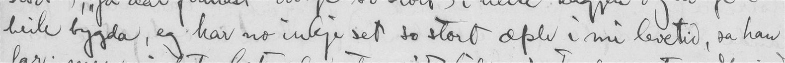heile bygda, og har no inkje sett so stort æple i mi levetid, sa han
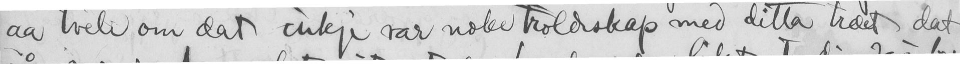aa tvele om dat inkje var noke troldskap med ditta træet dat
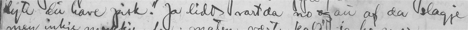lyte du have pisk." Ja lidt vart da no  au af da slagje
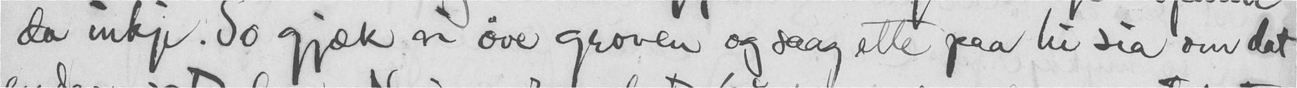de inkje! So gjæk vi øve groven og saag ette paa lu sia om det
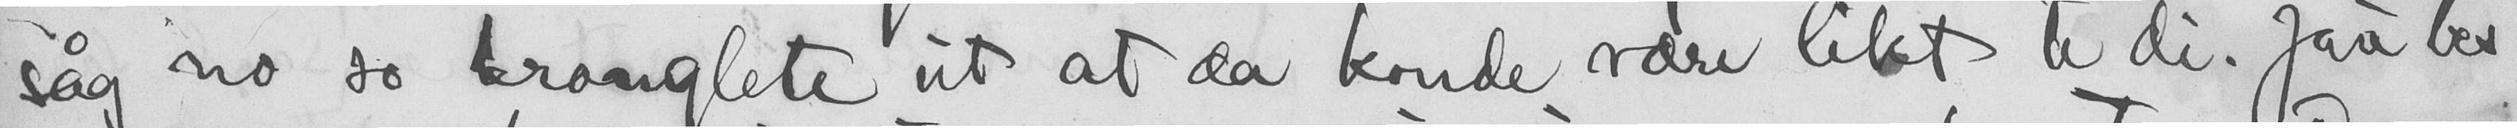såg no so kronglete ut at da konde være likt te de. Jau be
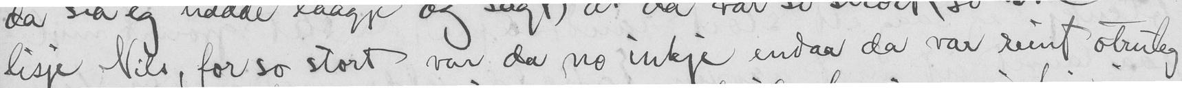lisje Nils, for so stort var da no inkje endaa da var reint otruleg
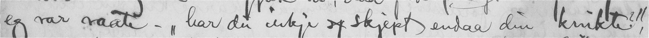eg var vaate - "har du ikkje  skjept endaa din krukte?",
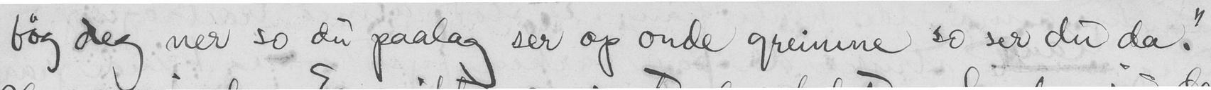bøg deg ner so du paalag ser op onde greinene so ser du da."
